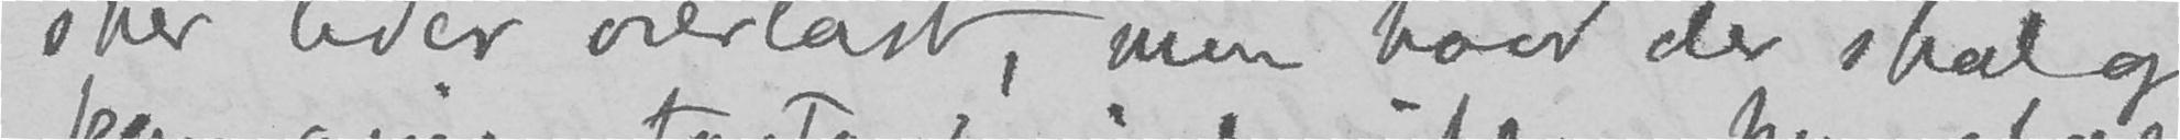sker lider overlast, men hvad der skal og
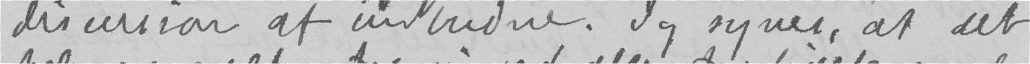discussion af indbudne. Jeg synes, at det
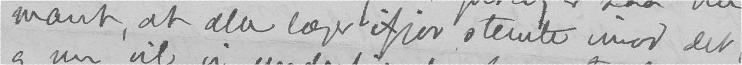mant, at alle læger ifjor stemte imod det,
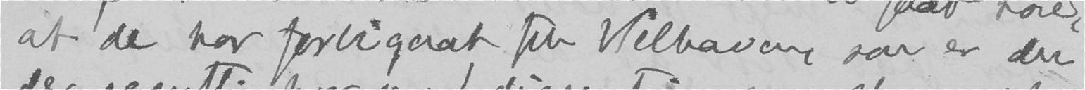at de har forbigaat frk Welhaven, som er den
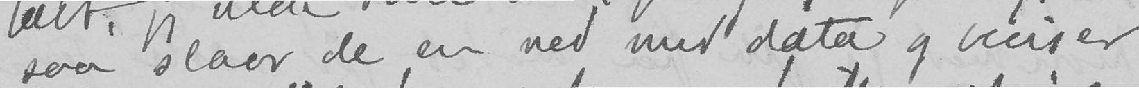saa slaar de en ned med data og beviser
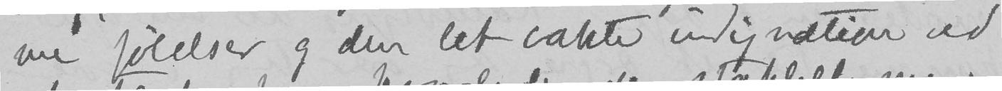me følelser og den let vakte indignation ved
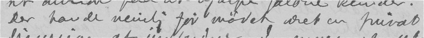Der havde nemlig før mødet været en privat
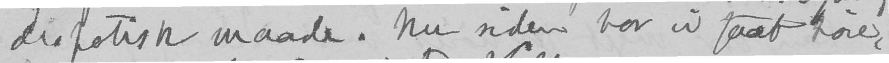despotisk maade. Nu siden har vi faat høre,
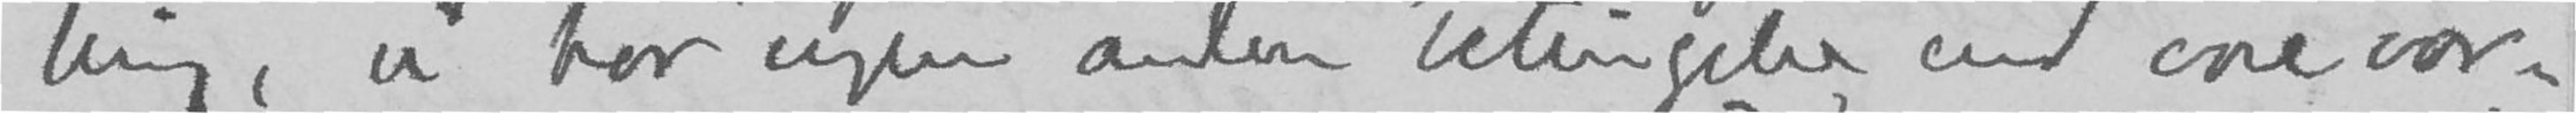ting, vi har ingen anden betingelse end vore var-
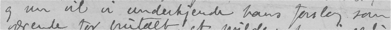og nu vil vi underkjende hans forslag som
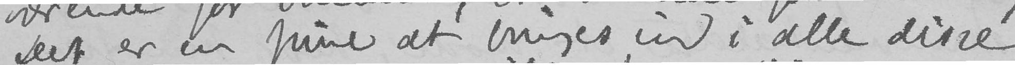Det er en pine at bringes ind i alle disse
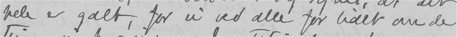hele er galt, for vi ved alle for lidet om de
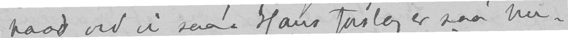hvad ved vi saa. Hans forslag er saa hu-
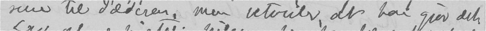reise til Jæderen, men betvivler, at han gjør det.
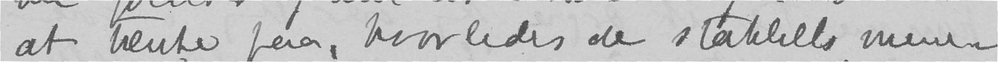at tænke paa, hvorledes de stakkels menne-
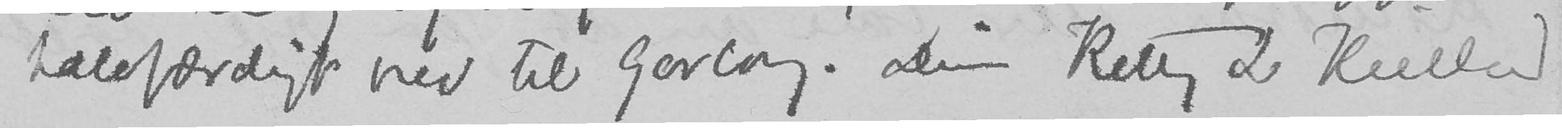halvfærdigt brev til Garborg. Din Kitty L Kielland
